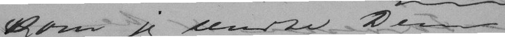som jeg sendte Dem
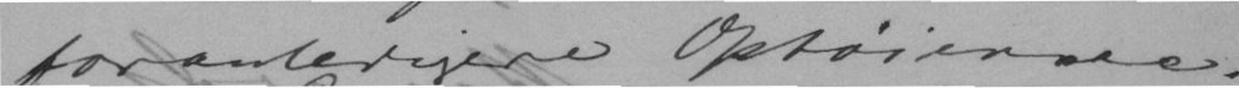foranledigere Optøierne.
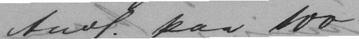Anvf. paa 100
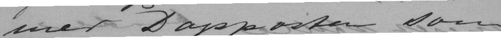med Dagsposten som
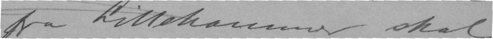fra Lillehammer skal
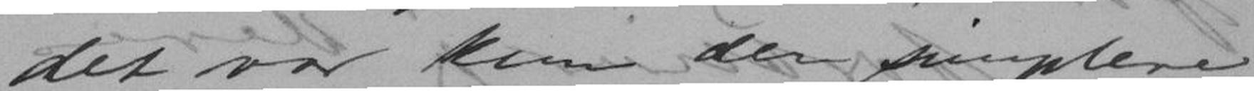det var kun der simplere
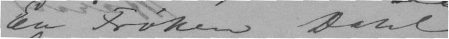En Frøken Dahl
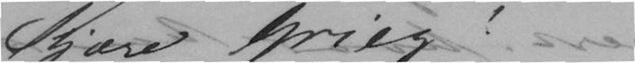Kjære Grieg!
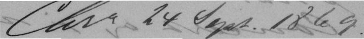Chr. 24 Sept. 1869.
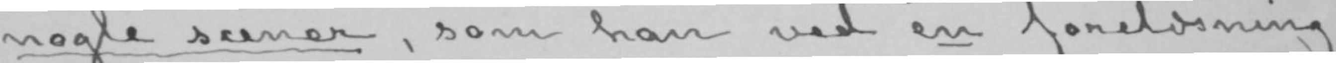nogle scener, som han ved en forelæsning
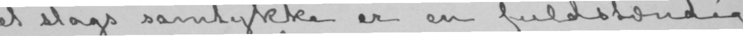et slags samtykke er en fuldstændig
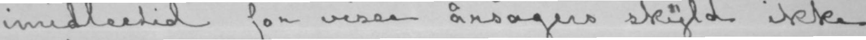imidlertid for visse årsagers skyld ikke
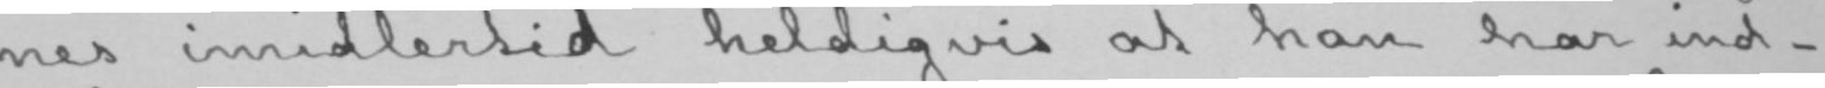nes imidlertid heldigvis at han har ind-
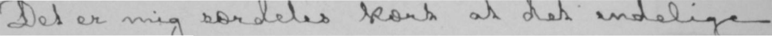Det er mig særdeles kært at det endelige
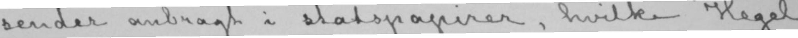sender anbragt i statspapirer, hvilke Hegel
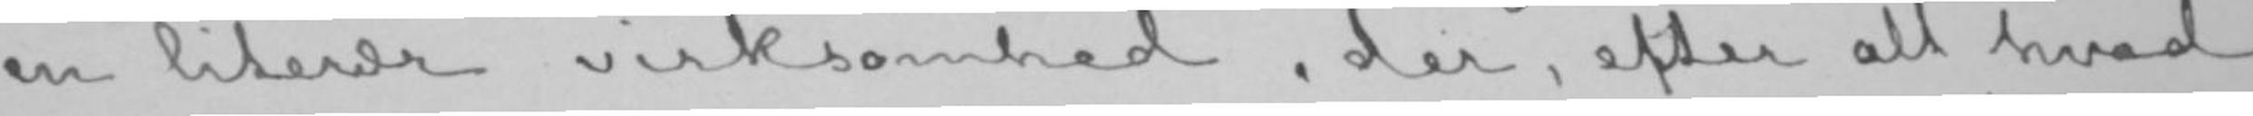en literær virksomhed, der, efter alt hvad
